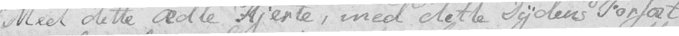Med dette ædle Hjerte, med dette Dydens Forsæt
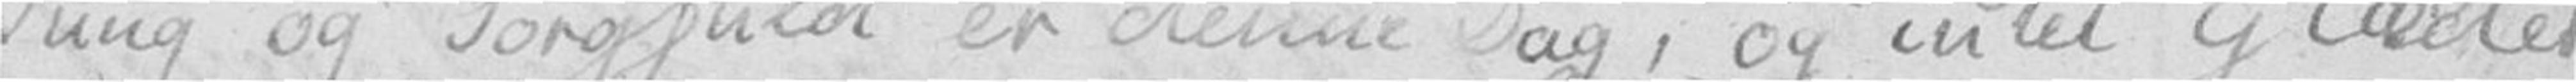Tung og Sorgfuld er denne Dag, og intet glæder
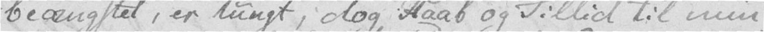beængstet, er tungt, dog Haab og Tillid til min
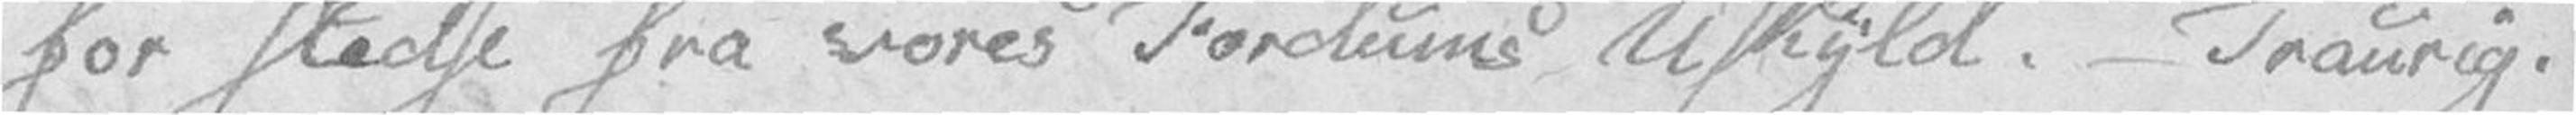for stedse fra vores Fordums Uskyld. – Traurig.
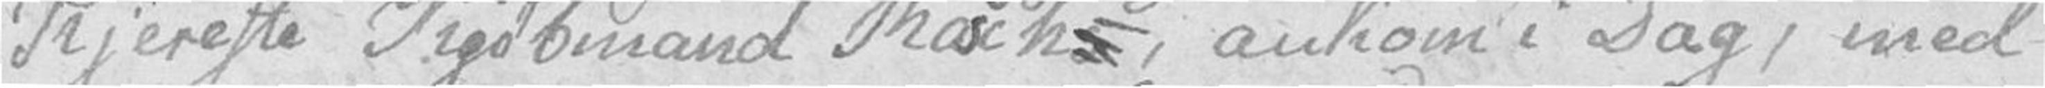Kjereste Kjøbmand Raschs, ankom i Dag, med
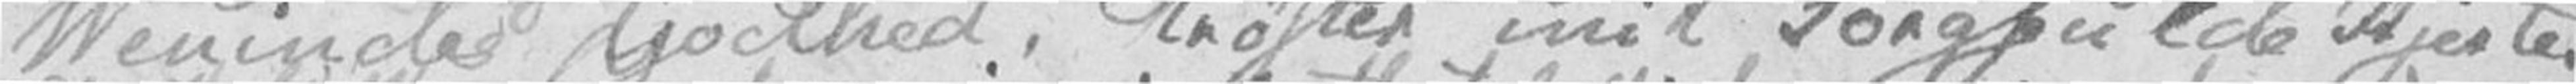Venindes Godhed, trøster mit Sorgfulde Hjerte.
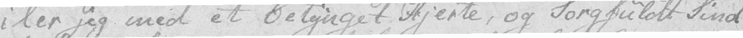iler jeg med et betynget Hjerte, og Sorgfuldt Sind
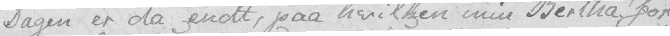Dagen er da endt, paa hvilken min Bertha! for
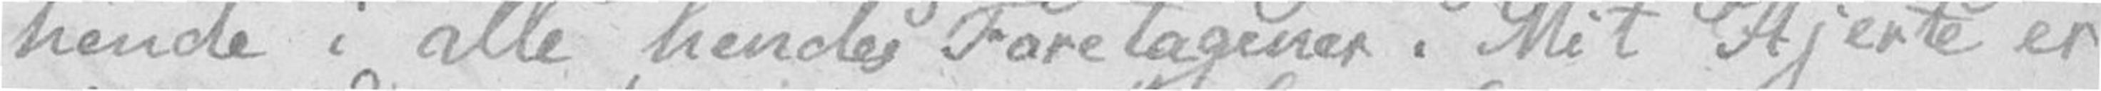hende i alle hendes Foretagener. Mit Hjerte er
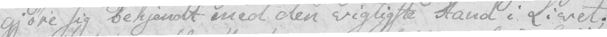gjøre sig bekjendt med den vigtigste Stand i Livet.
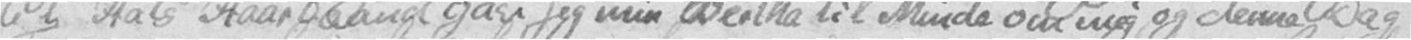Et Hals Haarbaand gav jeg min Bertha til Minde om mig og denne Dag.
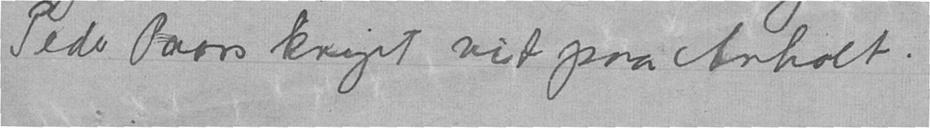Peder Paars kriget vist paa Anholt.
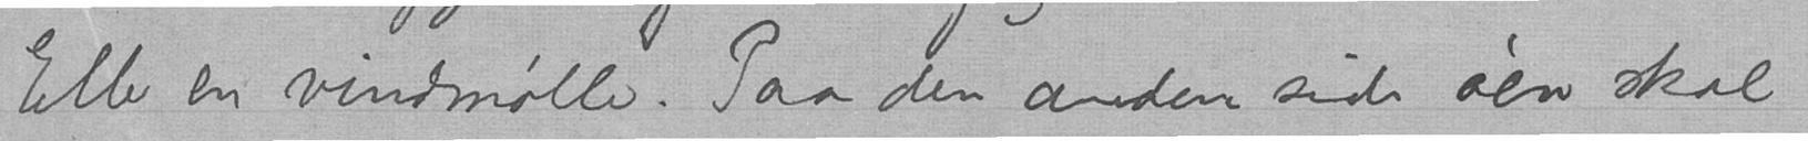Eller en vindmølle. Paa den anden side øen skal
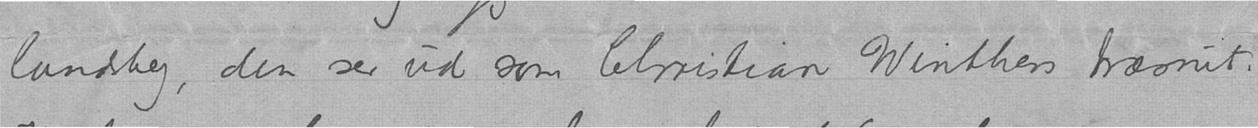landsby, den ser ud som Christian Winthers træsnit.
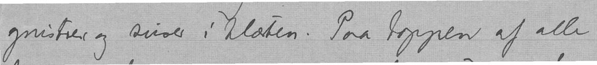gnistrer og suser i blæsten. Paa toppen af alle
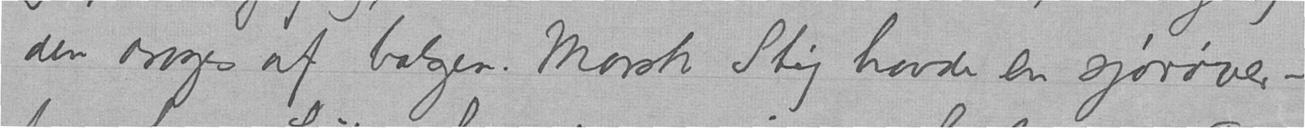den drages af bælgen. Marsk Stig havde en sjørøver-
